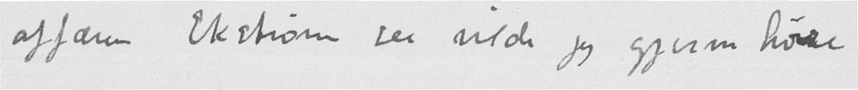affæren Ekstrøm saa vilde jeg gjerne høre
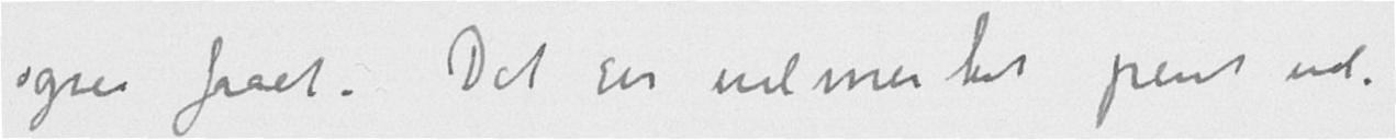ogsaa faaet. Det ser udmerket pent ud.
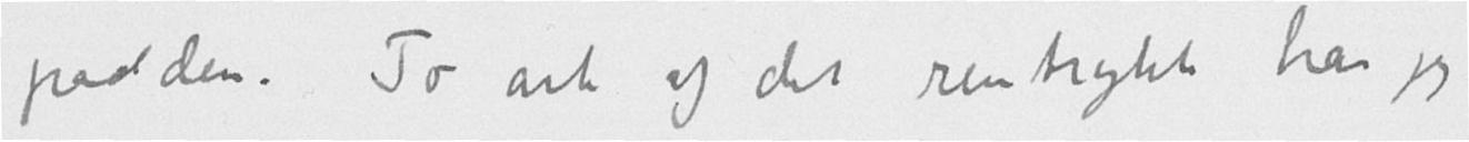padden. To ark af det rentrykte har jeg
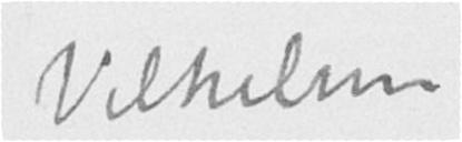Vilhelm
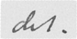det.
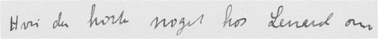Hvis du hørte noget hos Lenard om
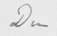Din
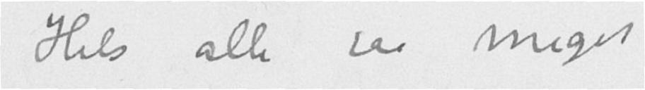Hils alle saa meget
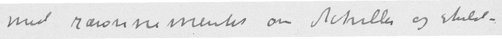med ræsonnementet om Achilles og skild-
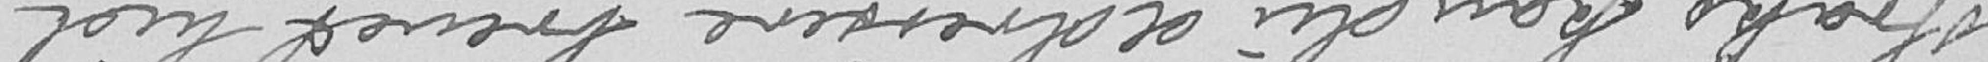straks kan du adressere brevet hid
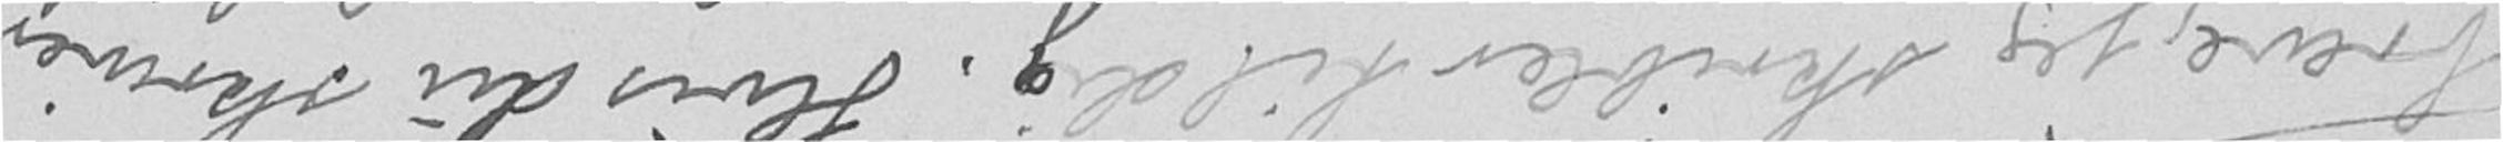breve jeg skribler til dig. Hvis du skriver
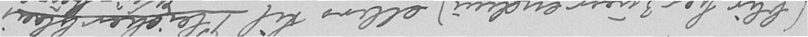(blir her 3 uger endnu) ellers til Pleicher Glacis
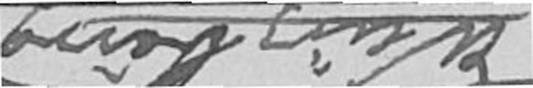Würzburg
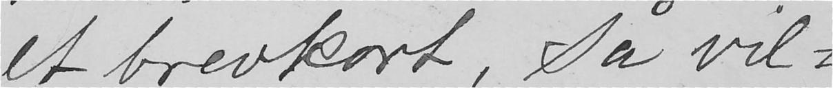et brevkort, så vil-
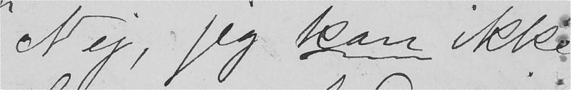Nej, jeg kan ikke
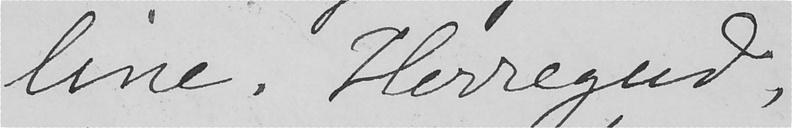line. Herregud,
